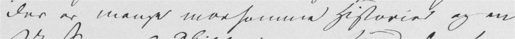der er mange morsomme Historier og en
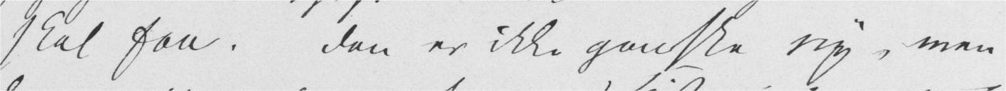skal faa. Den er ikke ganske ny, men
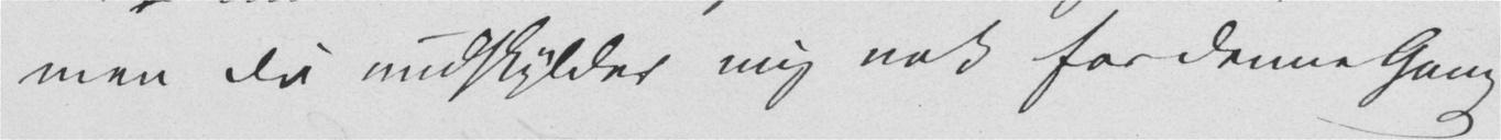men Du unskylder mig nok for denne Gang
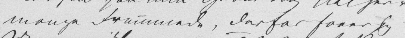mange Fremmede, derfor faaer jeg i
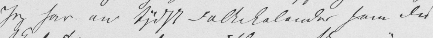Jeg har en tydsk Folkekalender som Du
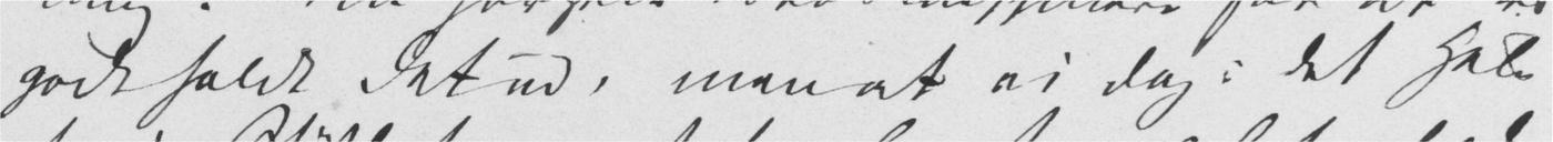godt holdt det ud, men at vi dog i det Hele
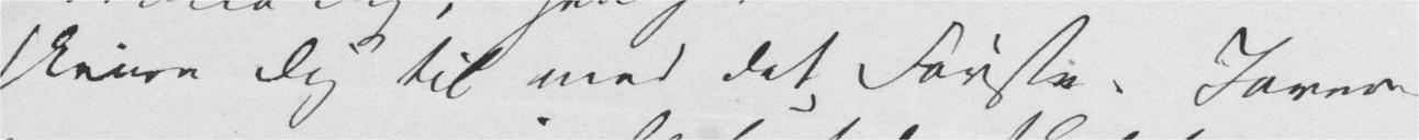skrive Dig til med det Første. Iover-
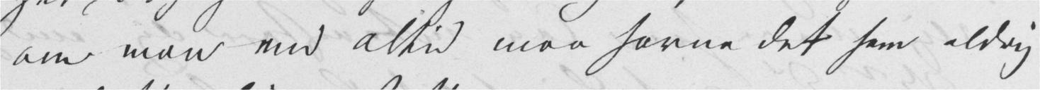om man end altid maa savne det som aldrig
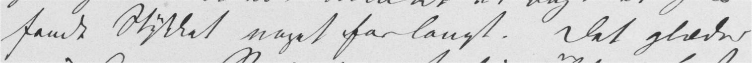fandt Stykket noget for langt. Det glæder
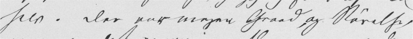selv. Der var megen Graad, og Rørelse,
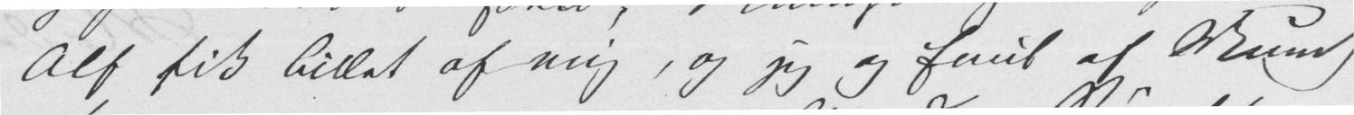Alf fik Billet af mig, og jeg og Emil af Munch
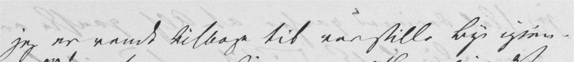jeg er vendt tilbage til vor stille By igjen.
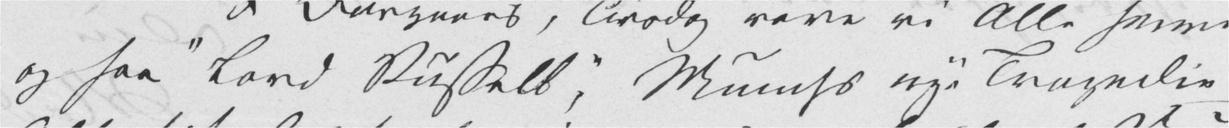og saa «Lord Russell», Munchs nye Tragedie,
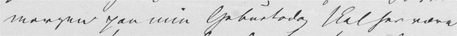morgen paa min Geburtsdag skal her være
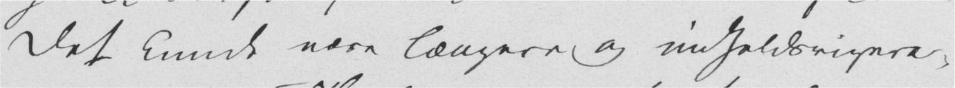Det kunde være længere og indholdsrigere,
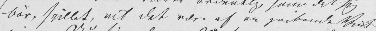bør, spillet, vil det være af en gribende Virk-
